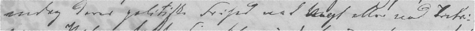endog deres politiske Frihed ved Magt eller ved Intri-
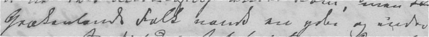Grækenlands Folk vandt en ydre og indre
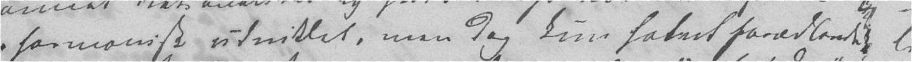harmonisk udviklet, men dog kun falnet forædlende
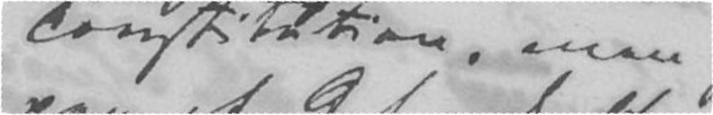Constitution, men
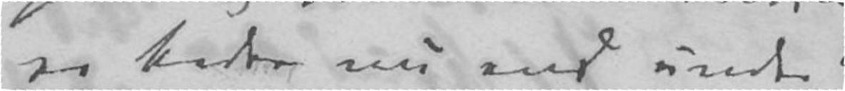er bedre nu end under
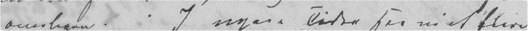overlegen. I nyere Tider see vi at flere
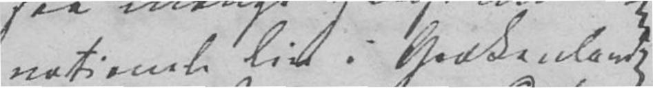nationale Liv i Grækenland
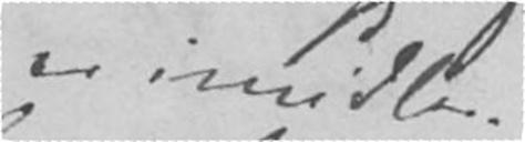er imidler-
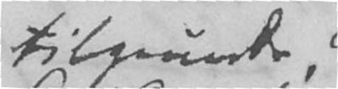tilgrunde,
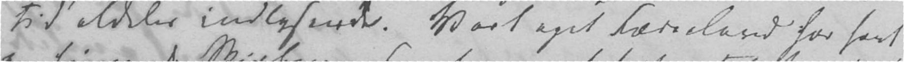tid aldeles indlysende. Vort eget Fædreland har havt
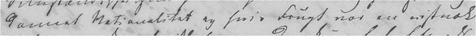dannet Nationalitet og hvis Frugt var en vistnok
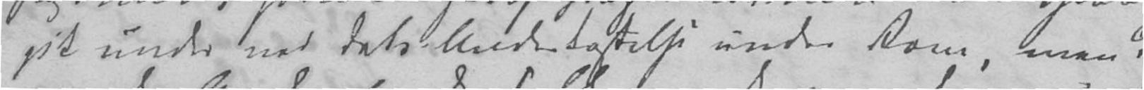gik under ved dets Underkastelse under Rom, men
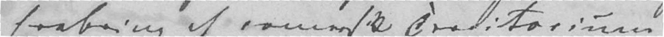Erobring af romersk Territorium
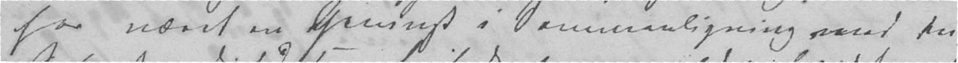har været en Gevinst i Sammenligning med den
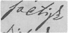factisk
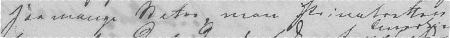saa mange Stater, men Privatretten
